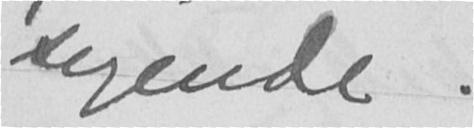sigende.
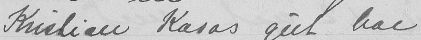Kristian Kasas gut har
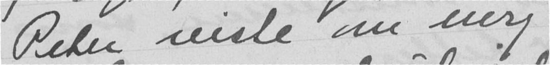Peter reiste om mrg.
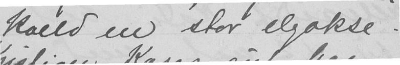kveld en stor elgokse.
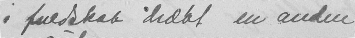i fuldskab dræbt en anden
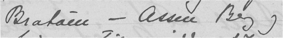Bratøen - Assen Berg og
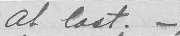At last. -
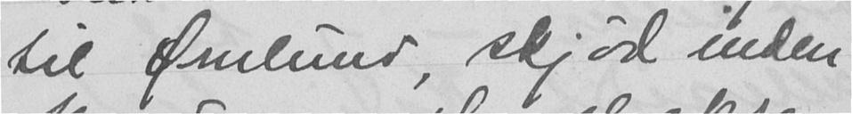til Ørnlund, skjød inden
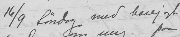16/9 Søndag med harejagt
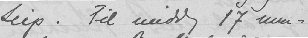Seip. Til middag 17 men-
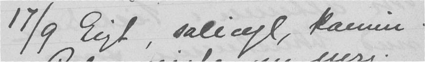17/9 Gigt, salicyl, kamin.
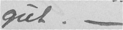gut. -
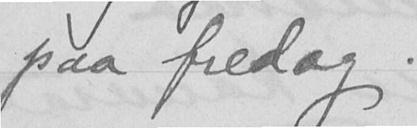paa fredag.
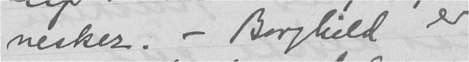nesker. - Borghild er
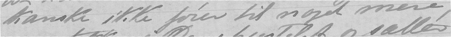kanske ikke fører til noget mere,
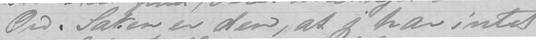Ord. Saken er den, at jeg har intet
